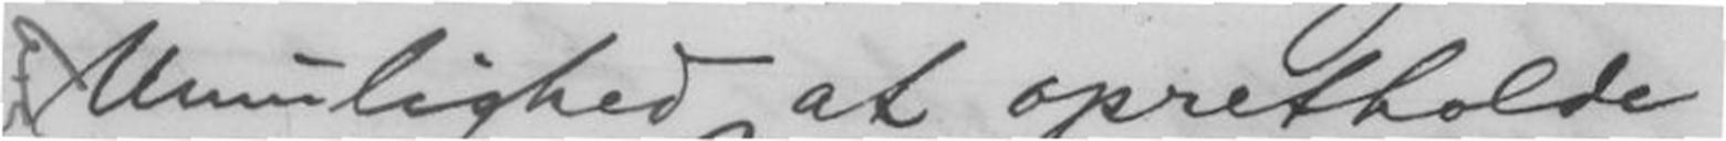Umulighed at opretholde
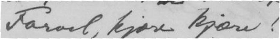Farvel, kjære kjære!
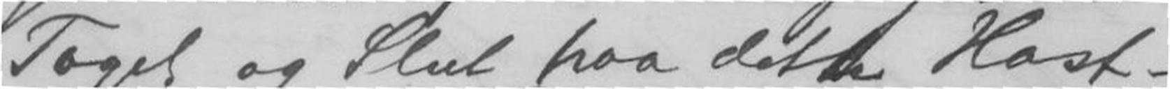Toget og Slut paa dette Hast-
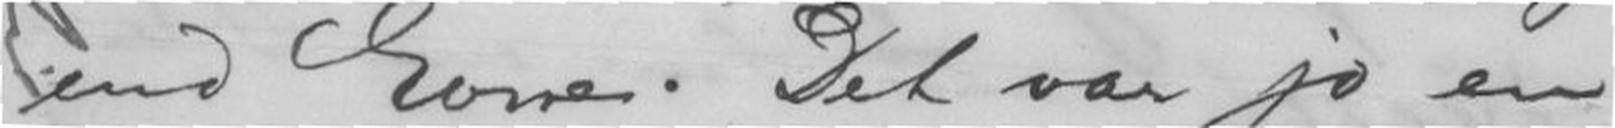end Evne. Det var jo en
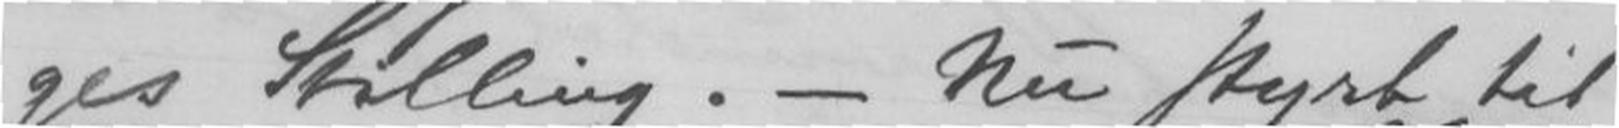ges Stilling. - Nu styrt til
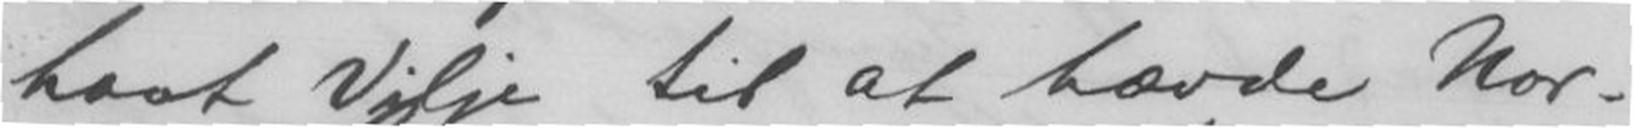havt Vilje til at hævde Nor-
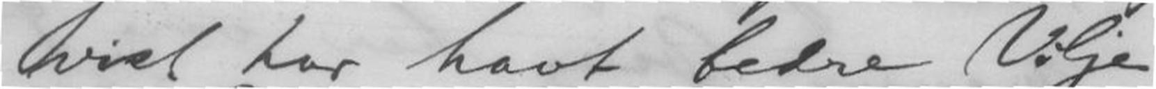vist har havt bedre Vilje
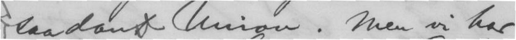saadan t Union. Men vi har
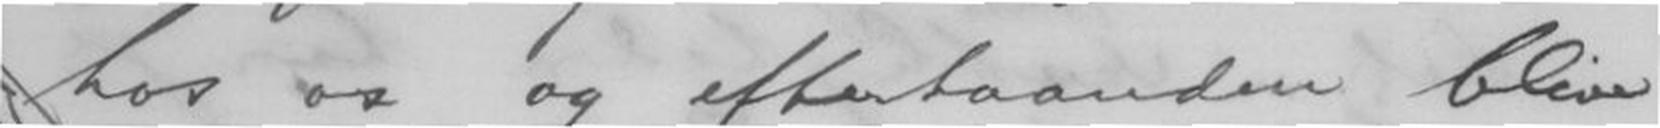hos os og efterhaanden blive
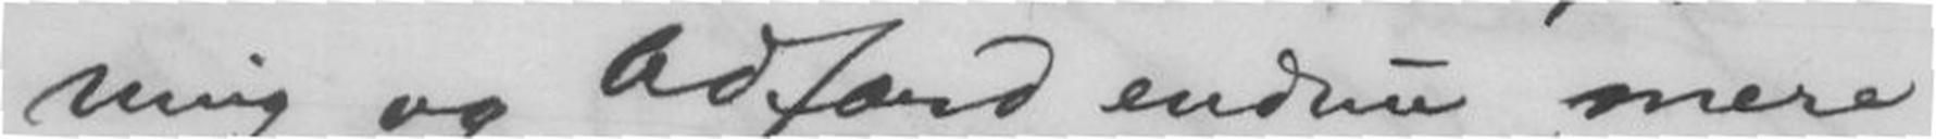ning og Adfærd endnu mere
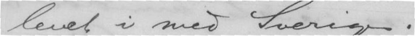har levet i med Sverige.
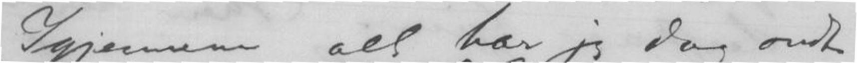Igjennen alt har jeg dog ondt
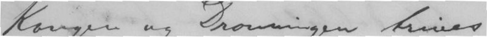Kongen og Dronningen trives
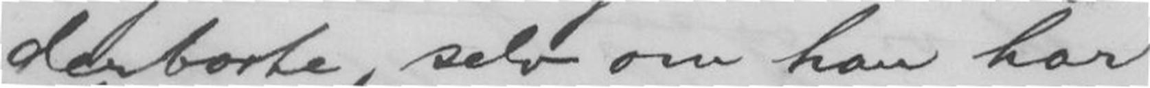derborte, selv om han har
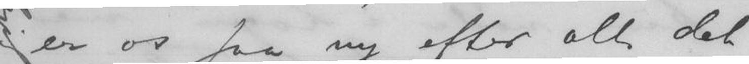er os saa ny efter alt det
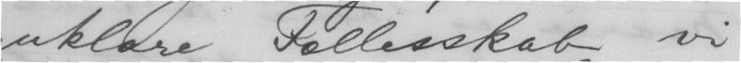uklare Fællesskab vi
